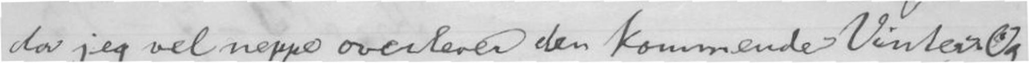da jeg vel neppe overlever den kommende Vinter, Og
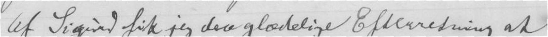Af Sigurd fik jeg den glædelige Efterretning at
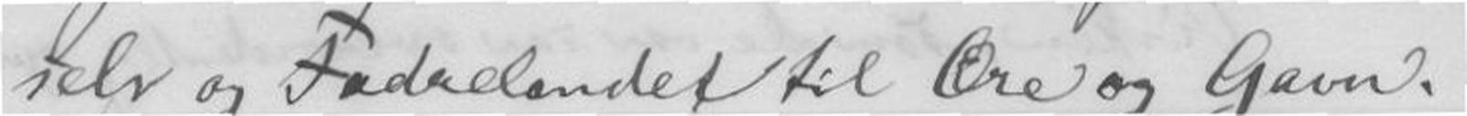selv og Fædrelandet til Ære og Gavn.
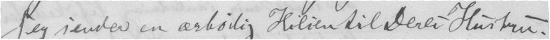Jeg sender en ærbødig Hilsen til Deres Hustru.
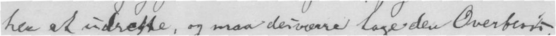her at udrette, og maa desværre tage den Overbevis-
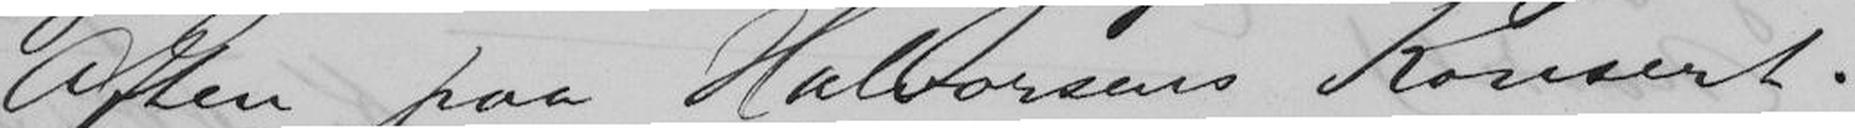Aften paa Halvorsens Konsert.
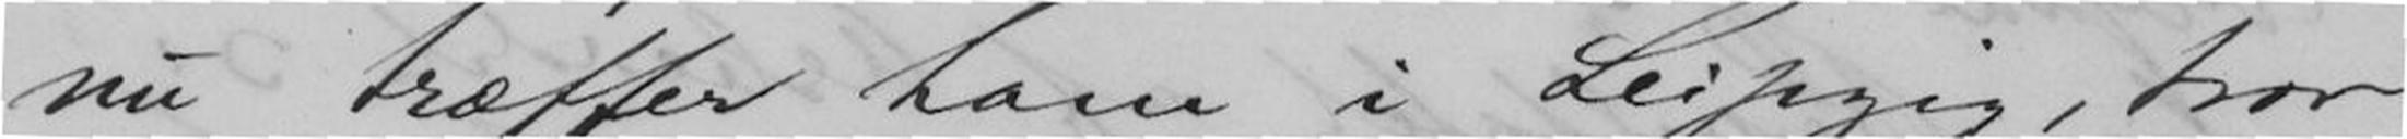nu træffer ham i Leipzig, tror
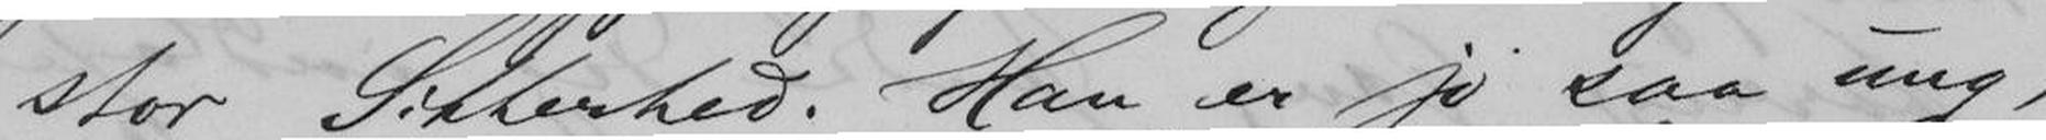stor Sikkerhed. Han er jo saa ung,
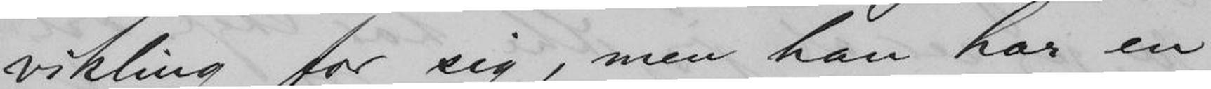vikling for sig, men han har en
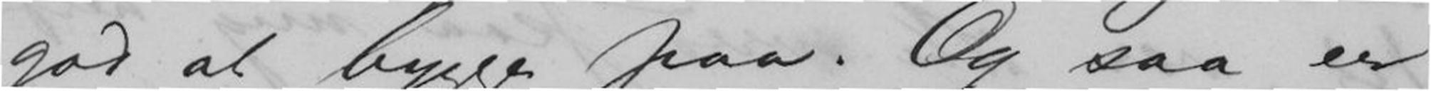god at bygge paa. Og saa er
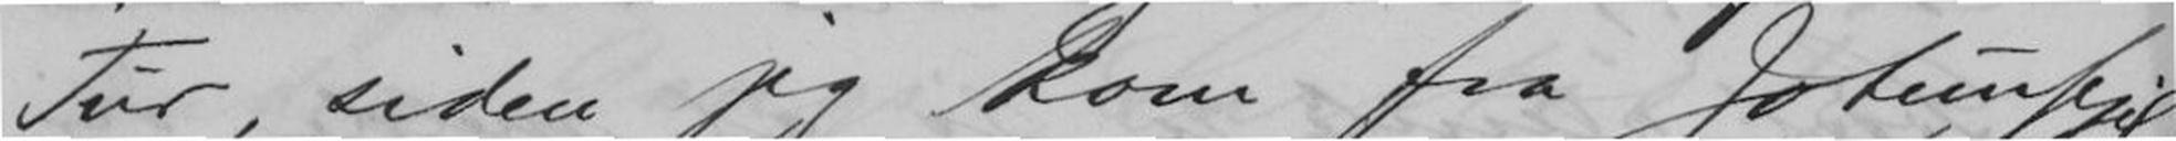Tur, siden jeg kom fra Jotunfjel-
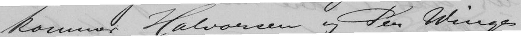kommer Halvorsen og Per Winges
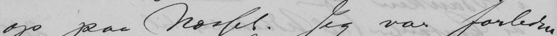op paa Næsset. Jeg var forleden
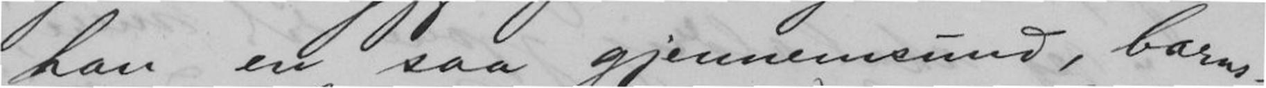han en saa gjennemsund, barns-
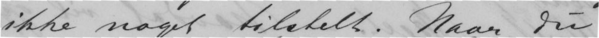ikke noget tilstelt. Naar du
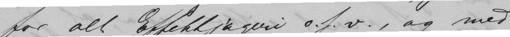for alt Effektjageri o.s.v., og med
